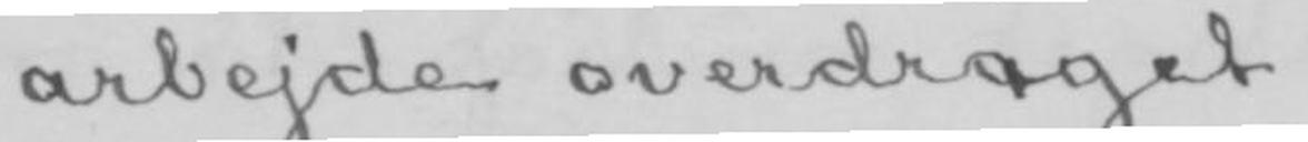arbejde overdraget.
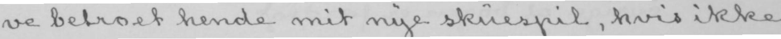ve betroet hende mit nye skuespil, hvis ikke
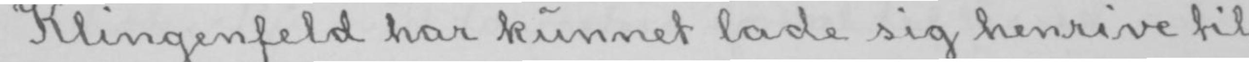Klingenfeld har kunnet lade sig henrive til
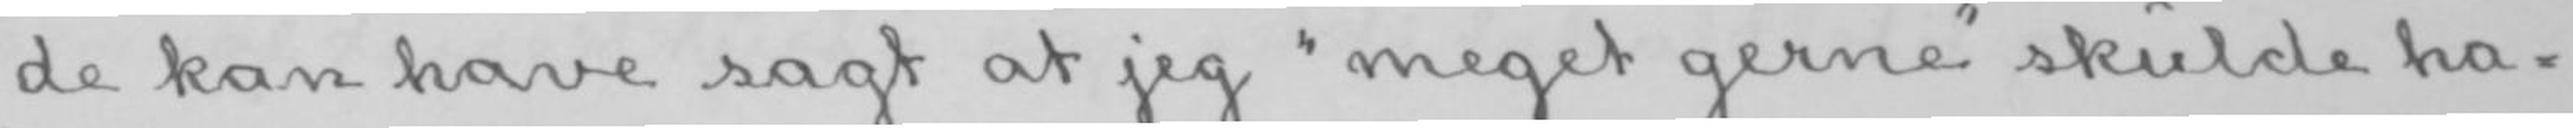de kan have sagt at jeg «meget gerne» skulde ha-
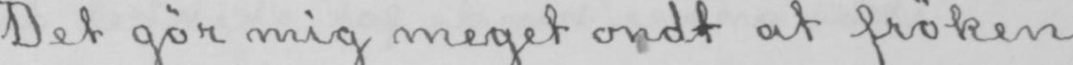Det gør mig meget ondt at frøken
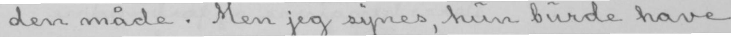den måde. Men jeg synes, hun burde have
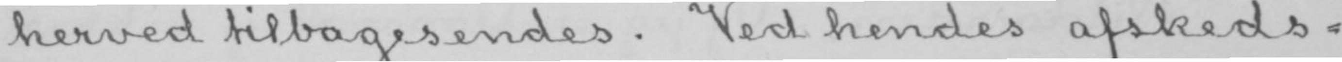herved tilbagesendes. Ved hendes afskeds-
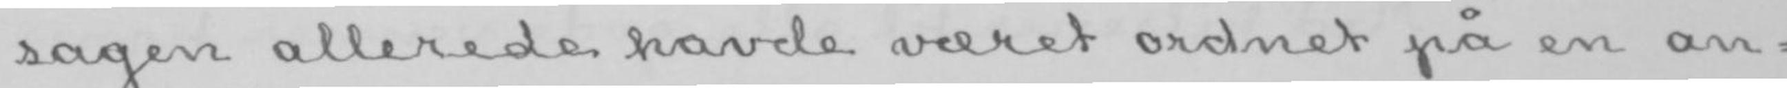sagen allerede havde været ordnet på en an-
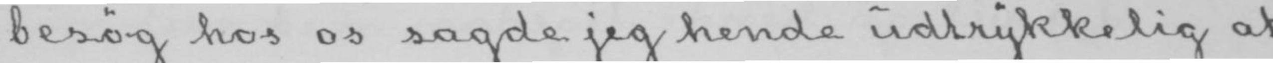besøg hos os sagde jeg hende udtrykkelig at
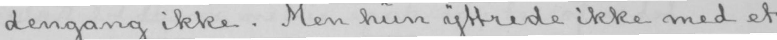dengang ikke. Men hun yttrede ikke med et
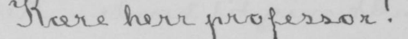Kære herr professor!
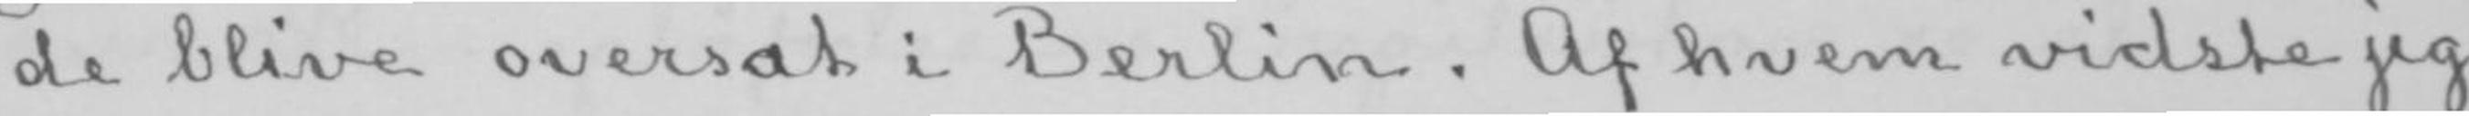de blive oversat i Berlin. Af hvem vidste jeg
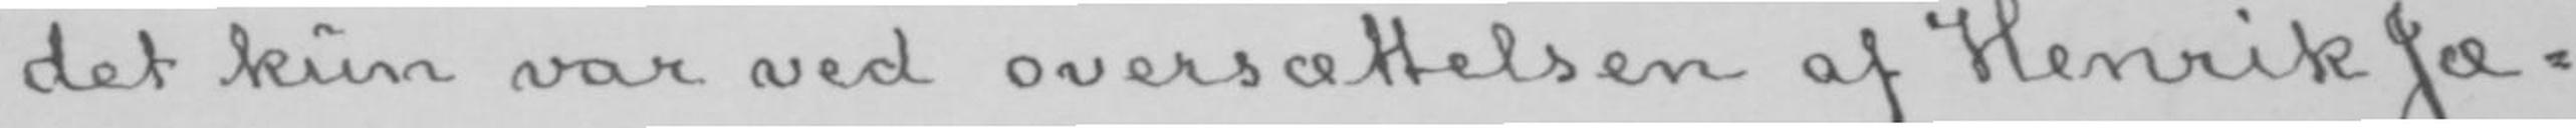det kun var ved oversættelsen af Henrik Jæ-
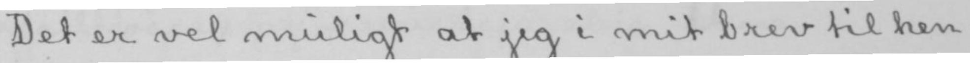Det er vel muligt at jeg i mit brev til hen-
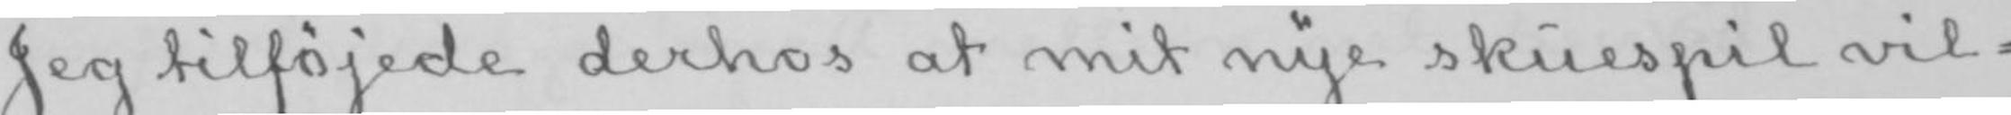Jeg tilføjede derhos at mit nye skuespil vil-
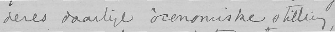deres daarlige øconomiske stilling,
